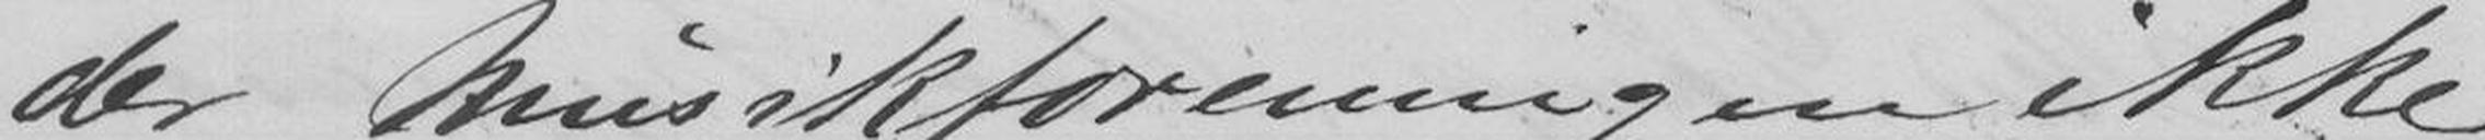der Musikforeningen ikke
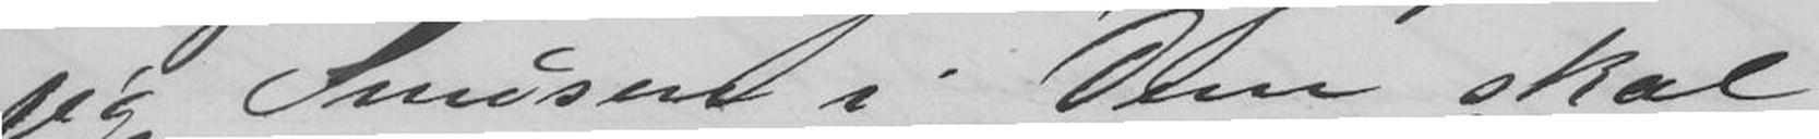jeg Snusen i dem skal
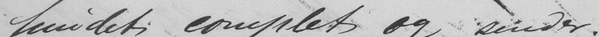fundet complet og sender.
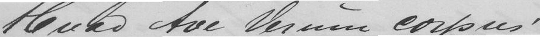Hvad Ave Verum corpus'
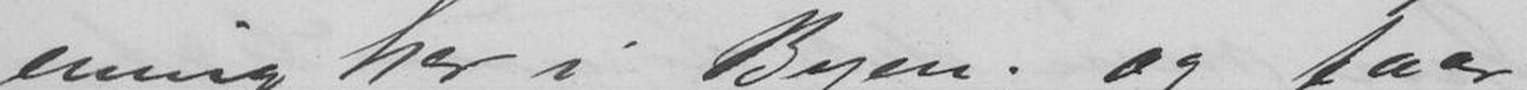ening her i Byen; og faar
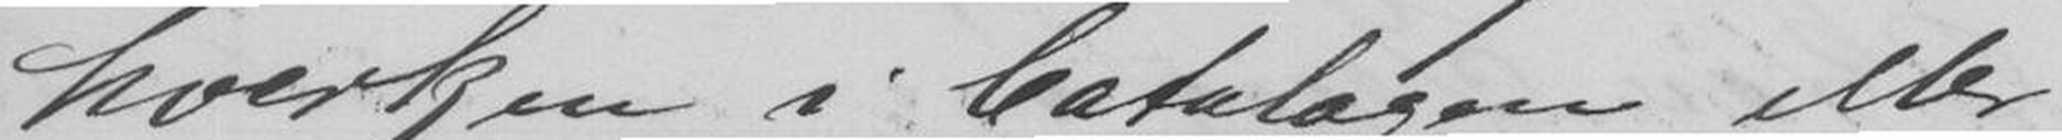hverken i Catalogen eller
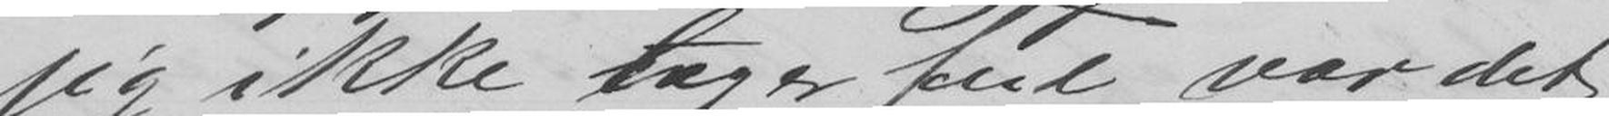jeg ikke tager Feil var det
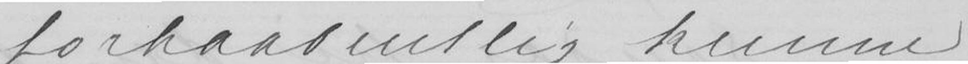forhaabentlig kunne
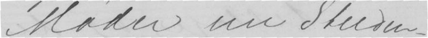Møder nu Studen-
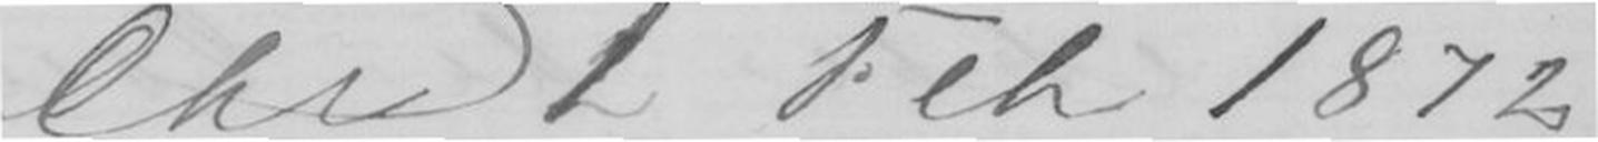Chr. 1 Feb. 1872
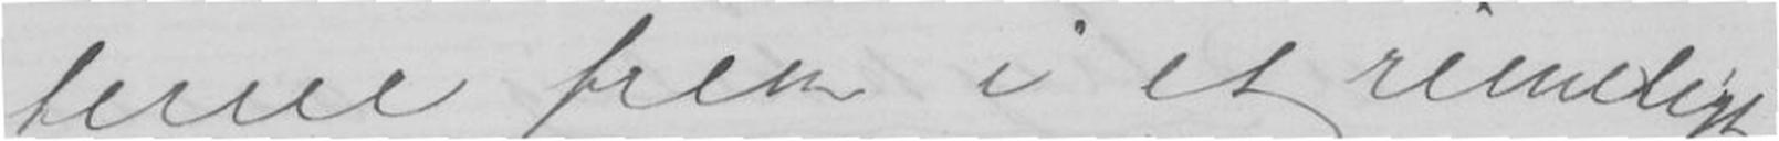terne frem i et rimeligt
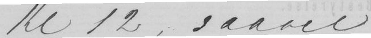Kl. 12, saavel
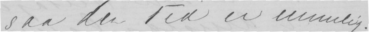saa, den Tid er umulig;
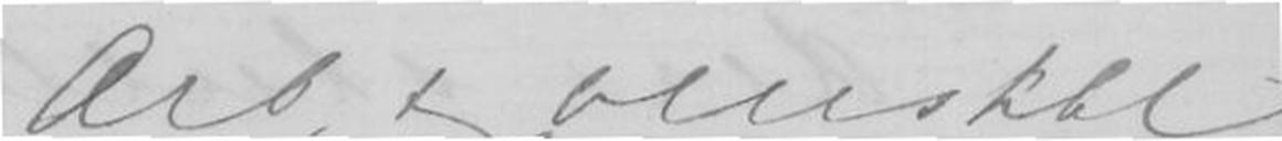Ærbtg venskbl
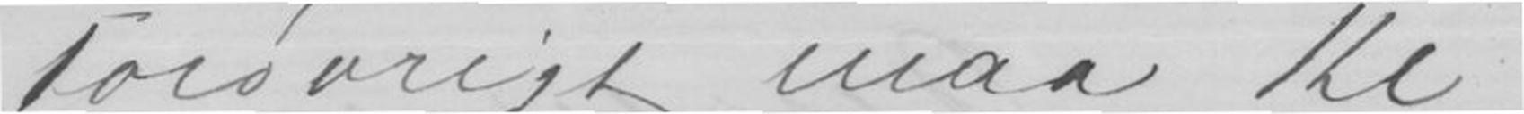Forøvrigt maa Kl 1
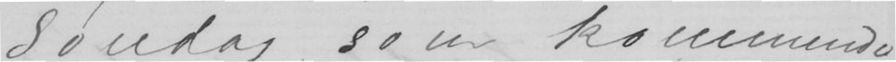Søndag som kommende
